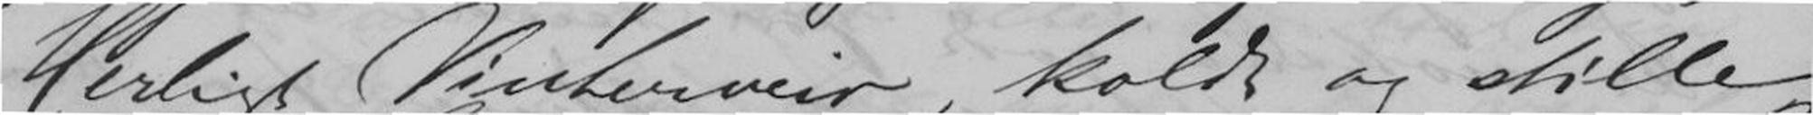Herligt Vinterveir, koldt og stille
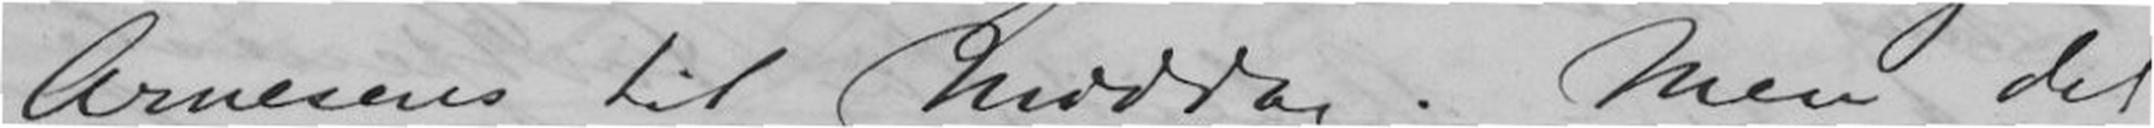Arnesens til Middag. Men det
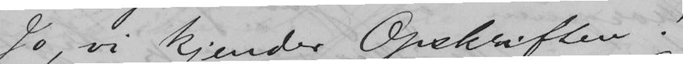Jo, vi kjender Opskriften!
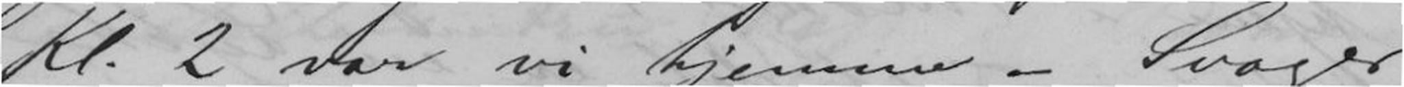Kl. 2 var vi hjemme - Svoger
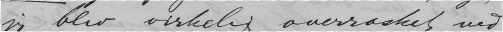jeg blev virkelig overrasket ved
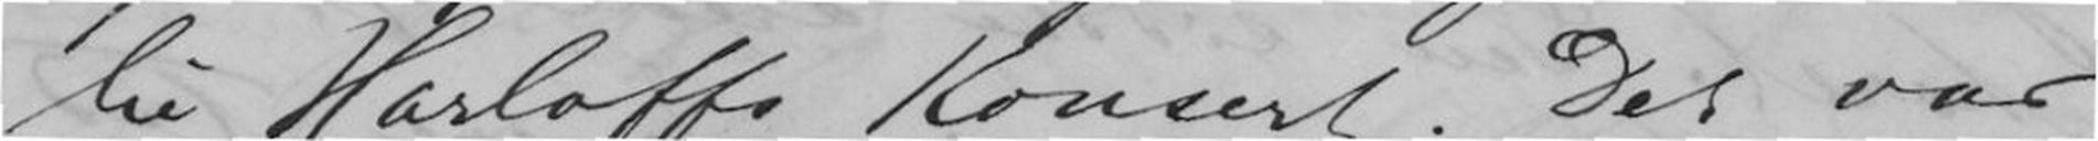lie Harloffs Konsert. Det var
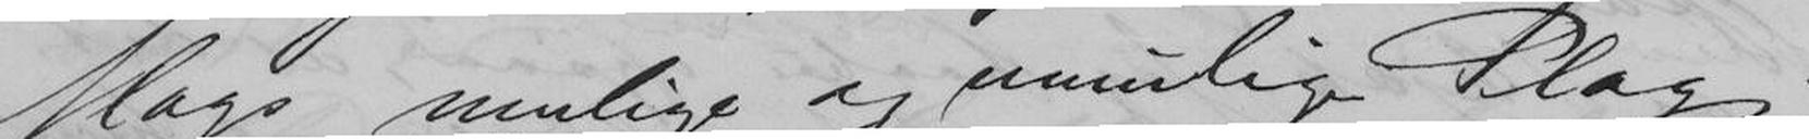slags mulige og umulige Plag.
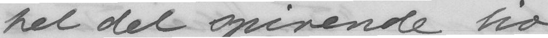hel del spirende tid
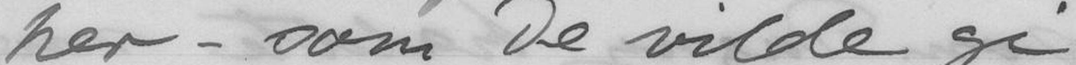her - som De vilde gi
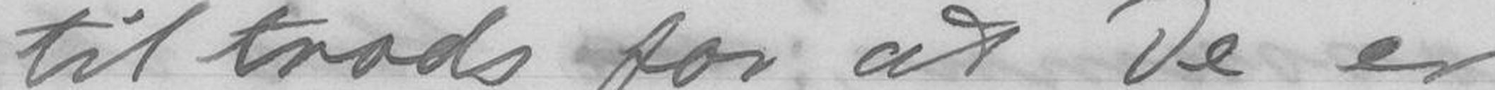tiltrods for at De er
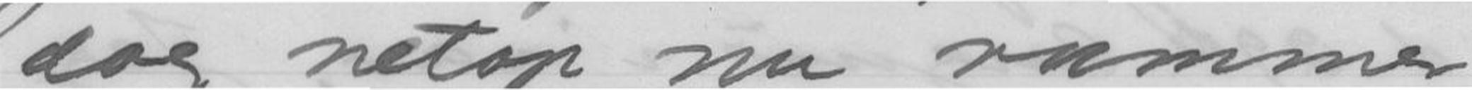dog netop nu rammer
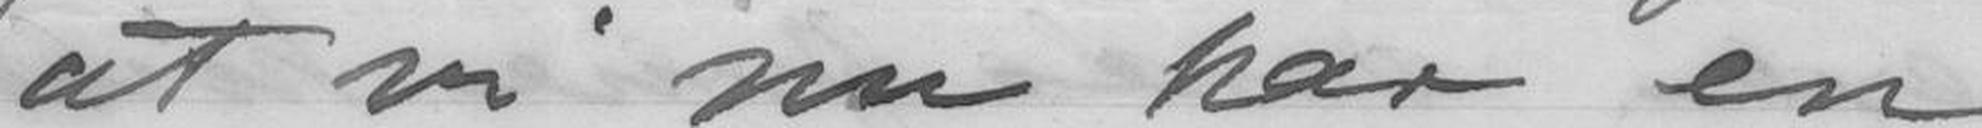at vi nu har en
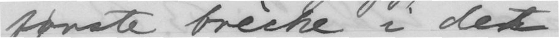første breche i det
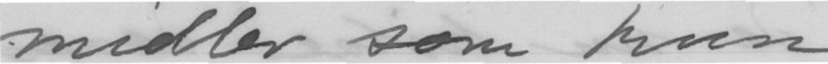midler som kun
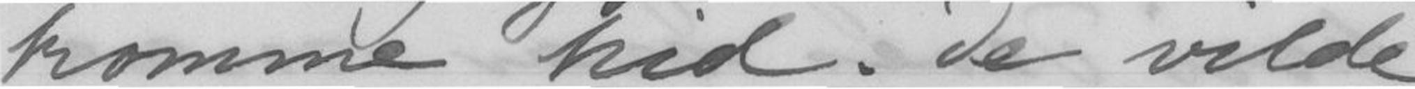komme hid. De vilde
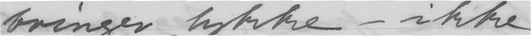bringer lykke - ikke
